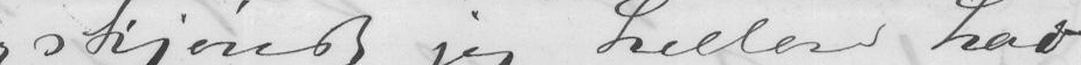skjøndt jeg heller hav
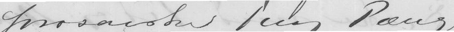prosaiske Ting Pænge
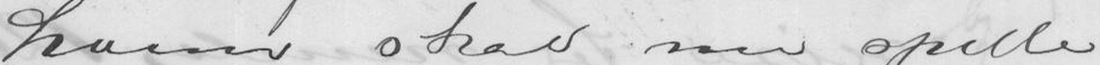hvem skal nu spille
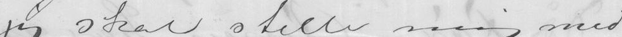jeg skal stille mig med
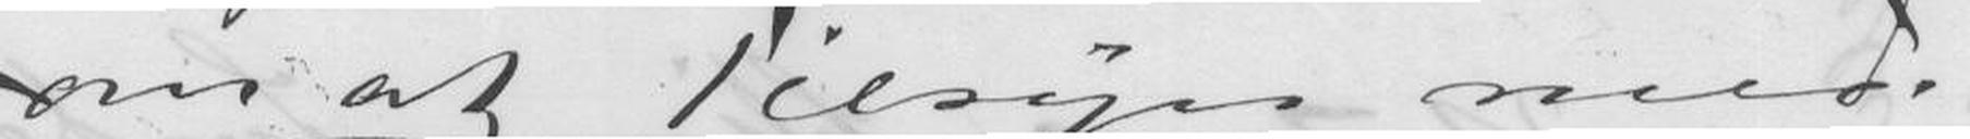om at Tilsyn med.
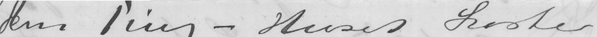den Ting - Huset koster
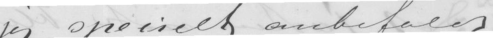jeg specielt anbefalet
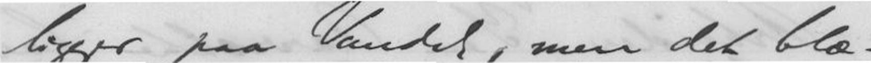ligger paa Vandet, men det blæ-
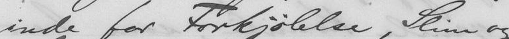inde for Forkjølelse, Slim og
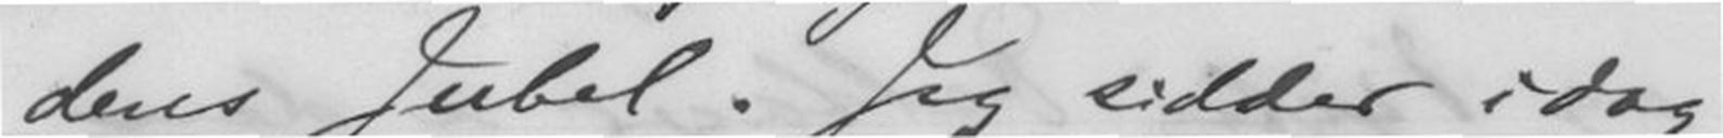deres Jubel. Jeg sidder idag
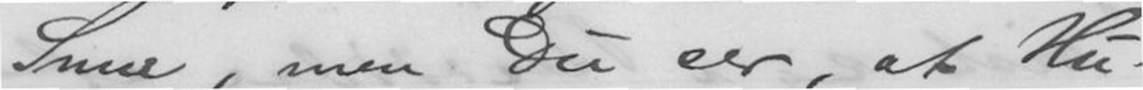Snue, men Du ser, at Hu-
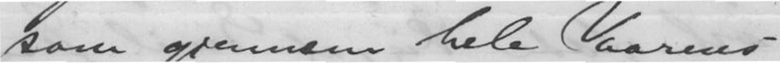som gjennem hele Vaarens
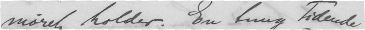møret holder. En tung Tidende
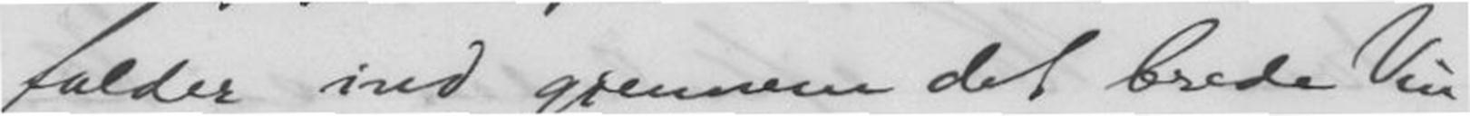falder ind gjennem det brede Vin-
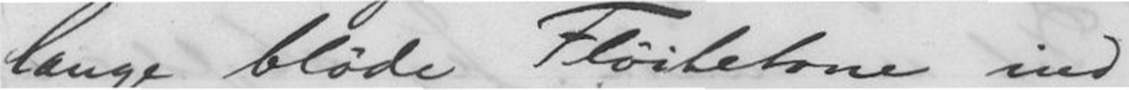lange bløde Fløitetone ind
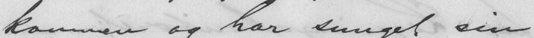kommen og har sunget sin
